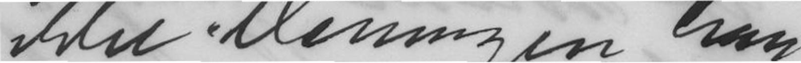ikke Meningen han
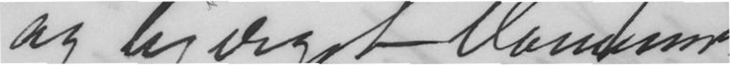og bjærget Monumentet
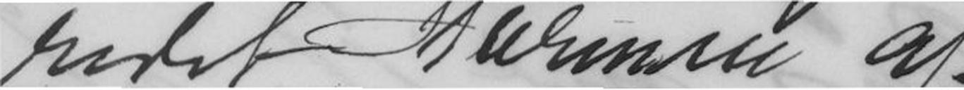redet Stormen af -
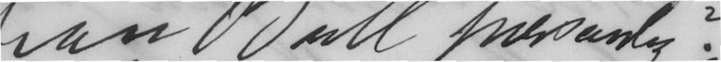han Bull personlig?
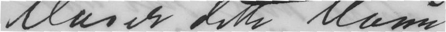Møier dette Monu
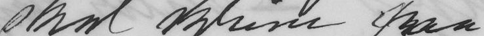skal skrive paa
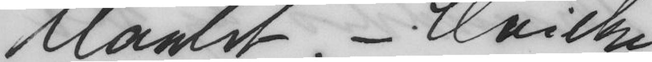Maalet. - Hvilke
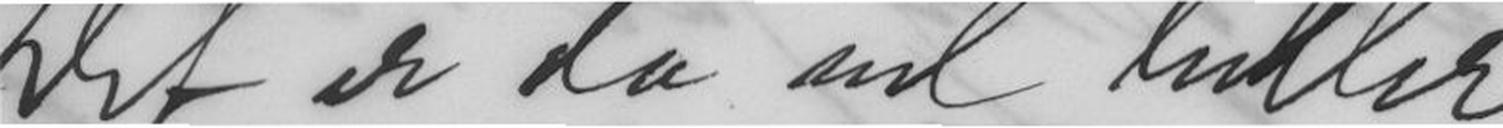Det er da vel heller
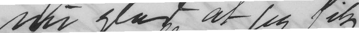nu glad at jeg fik
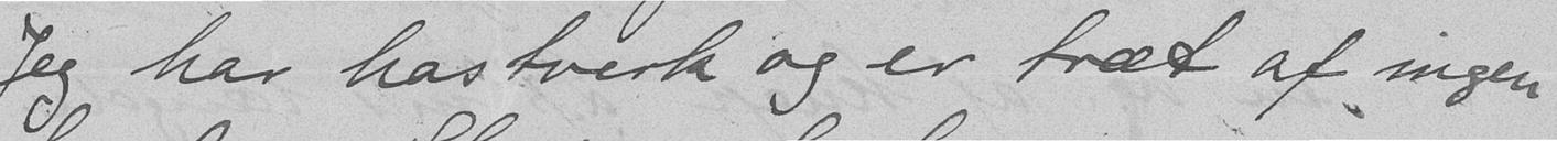Jeg har hastverk og er træt af ingen
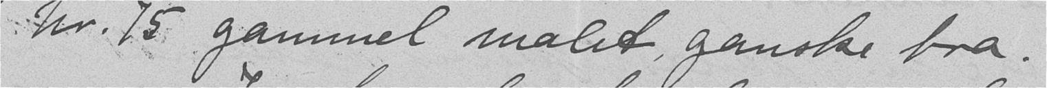Nr. 75 gammel malet, ganske bra.
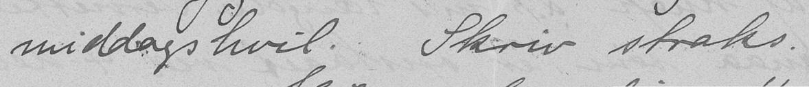middagshvil. Skriv straks.
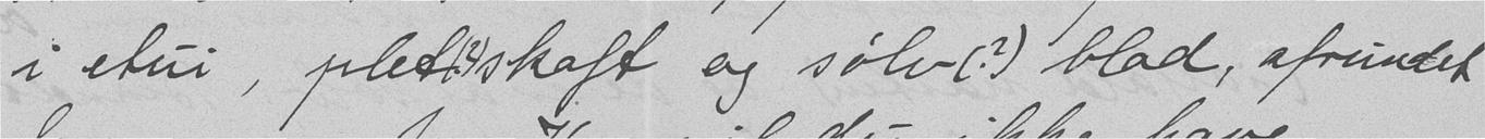i etui, plet(?)skaft og sølv(?) blad, afrundet
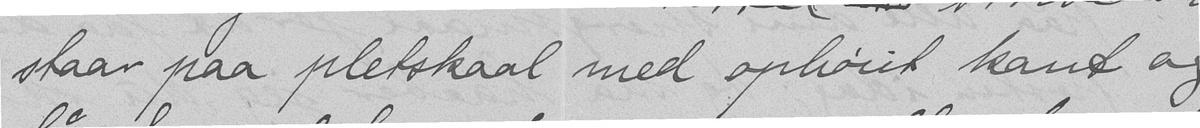staar paa pletskaal med ophøiet kant og
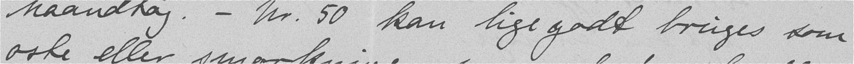haandtag. - Nr. 50 kan lige godt bruges som
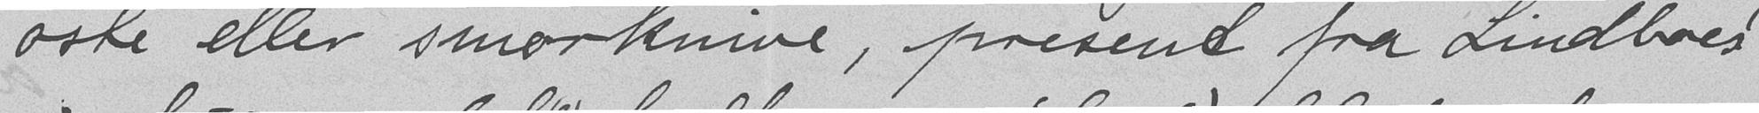oste eller smørknive, present fra Lindboes
